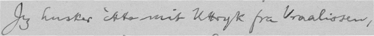Jeg husker ikke mit Uttryk fra Vraaliosen,
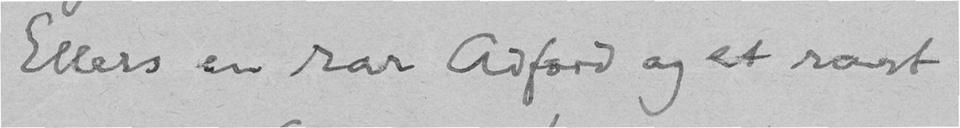Ellers en rar Adfærd og et rart
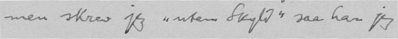men skrev jeg "uten Skyld" saa har jeg
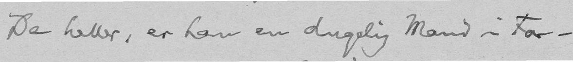De heller, er han en dugelig Mand i For-
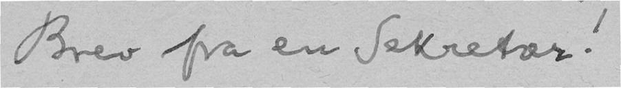Brev fra en Sekretær!
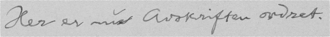Her er nu Avskriften ordret.
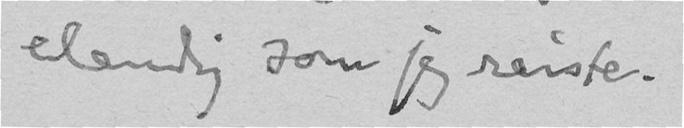elendig som jeg reiste.
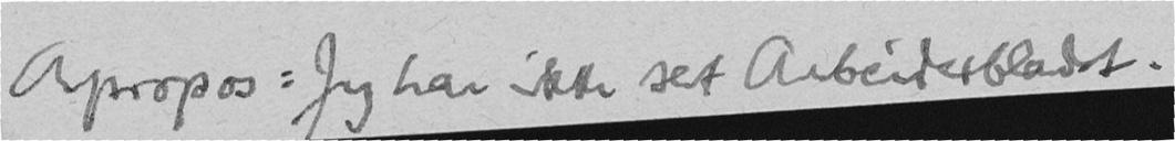Apropos: Jeg har ikke set Arbeiderbladet.
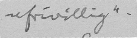ufrivillig".
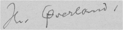Hr. Øverland,
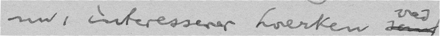nu, interesserer hverken dens
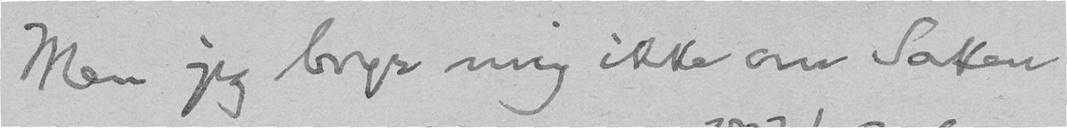Men jeg bryr mig ikke om Saken
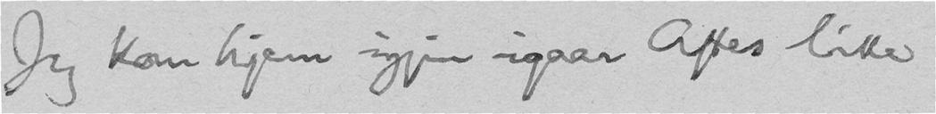Jeg kom hjem igjen igaar Aftes like
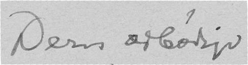Deres ærbødige
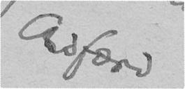Adfærd
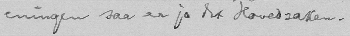eningen saa er jo det Hovedsaken.
